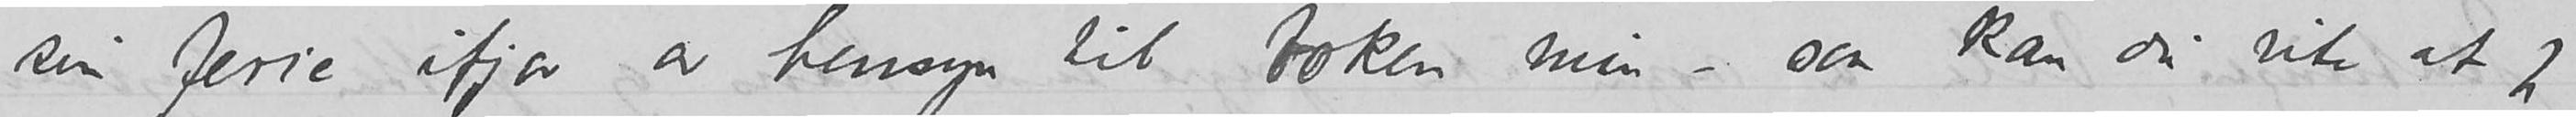sin ferie ifjor av hensyn til boken min – saa kan du vite at jeg
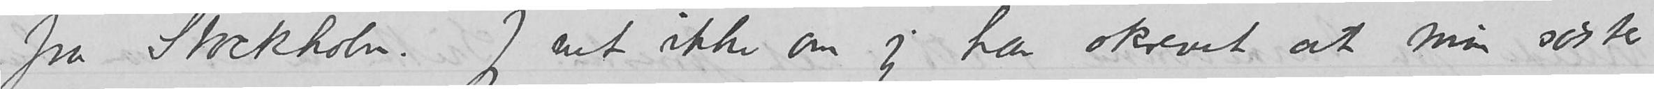fra Stockholm. Jeg vet ikke om jeg har skrevet at min søster
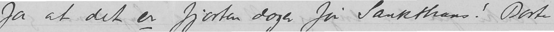Ja at det er fjorten dager før Sankthans! Borte
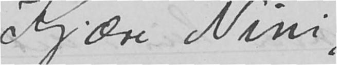Kjære Nini,
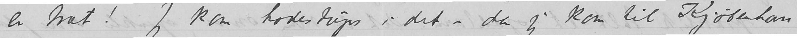er træt! Jeg kom hodestups i det – da jeg kom til Kjøbenhavn
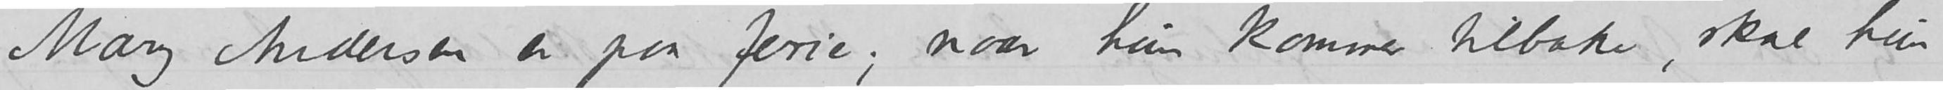Mary Andersen er paa ferie; naar hun kommer tilbake, skal hun
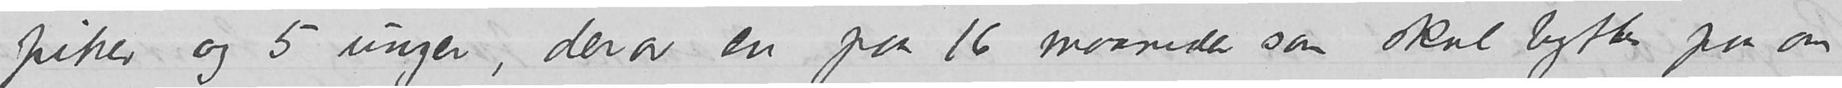piker og 5 unger, derav en paa 16 maaneder som skal byttes paa om
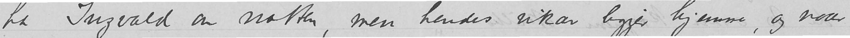ha Ingvald om natten, men hendes vikar ligger hjemme, og naar
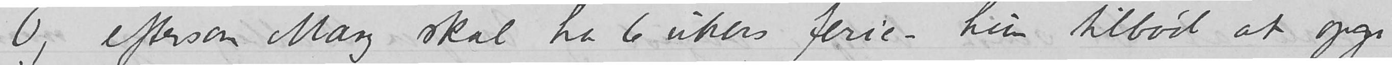Og eftersom Mary skal ha 6 ukers ferie – hun tilbød at opgi
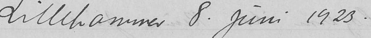Lillehammer     8. juni 1923
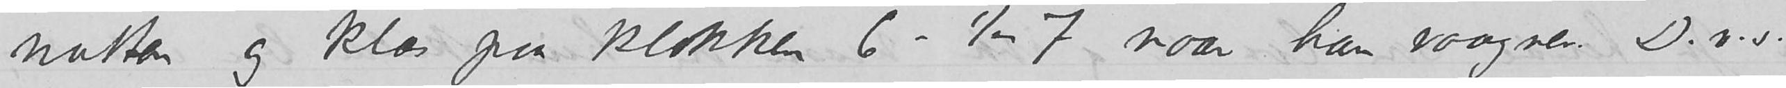natten og klæs paa klokken 6 -– ½ 7 naar han vaagner. D.v.s.,
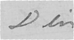Din
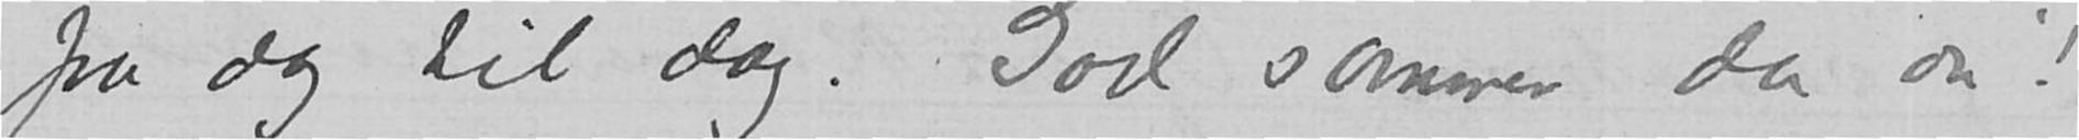fra dag til dag. God sommer da du!
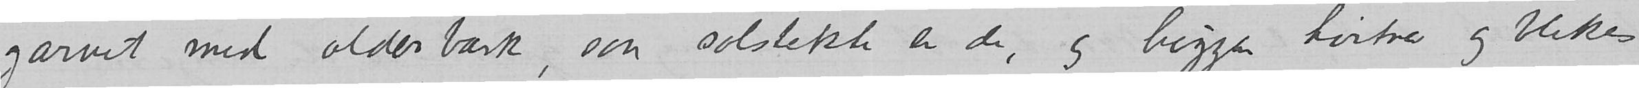garvet med olderbark, saa solstekte er de, og luggen hvitner og blekes
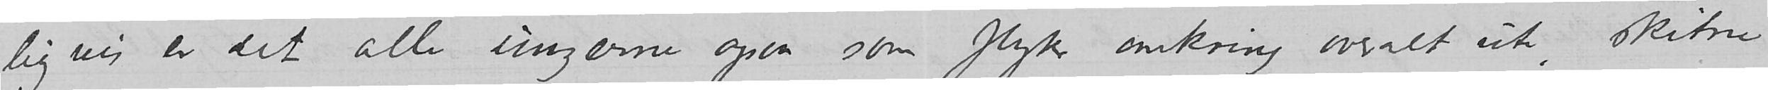ligvis er det alle ungerne ogsaa som flyter omkring overalt ute, skitne
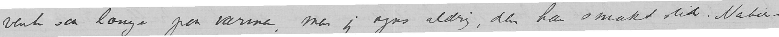vente saa længe paa varmen, men jeg syns aldrig, den har smakt slik. Natur
